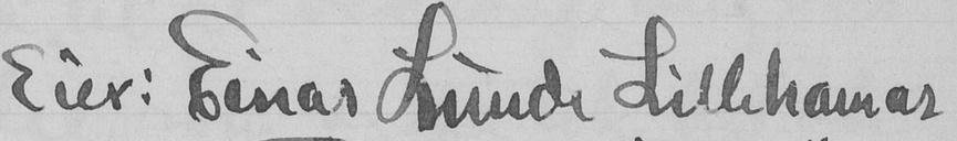Eier: Einar Lunde Lillehamar
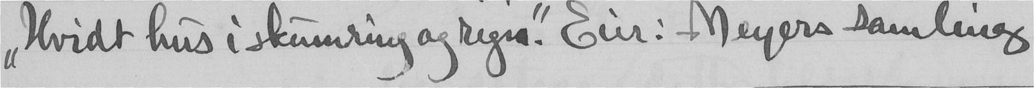"Hvidt hus i skumring og regn". Eier: Meyers samling
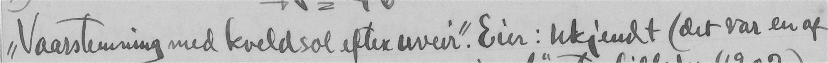"Vaarstemning med kveldsol efter uveir". Eier: ukjendt (det var en af
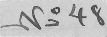No 48
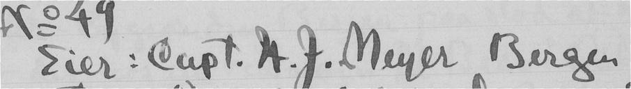Eier: Capt. H.J. Mayer Bergen
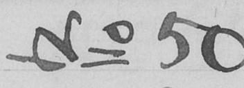No 50
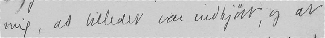mig, at billedet var indkjøbt, og at
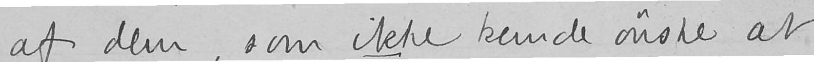af dem, som ikke kunde ønske at
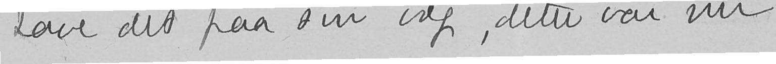have det paa sin væg, dette var nu
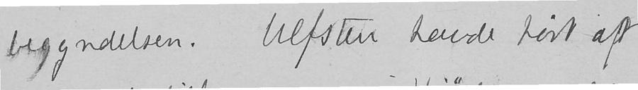begyndelsen. Ulfsten havde hørt af
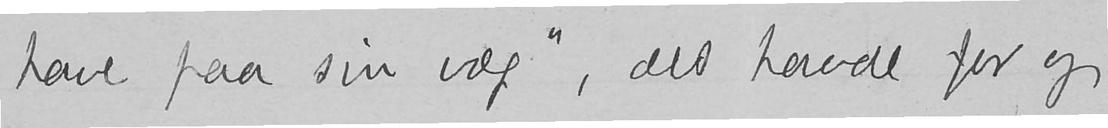have paa sin væg", det havde for og
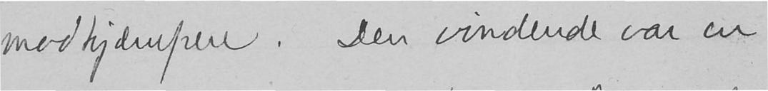modkjæmpere. Den vindende var en
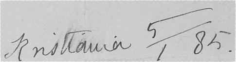Kristiania 5/1 85.
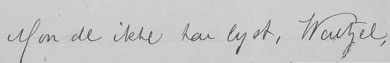Mon de ikke har lyst, Wentzel,
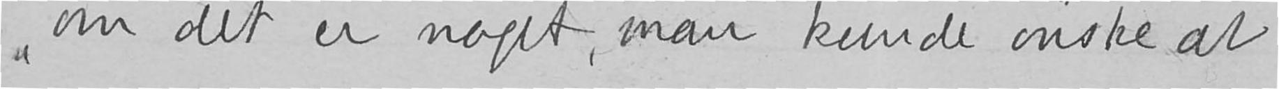"om det er noget, man kunde ønske at
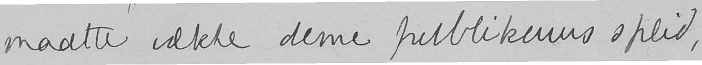maatte vække denne publikums splid,
